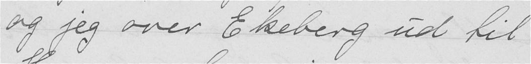og jeg over Ekeberg ud til
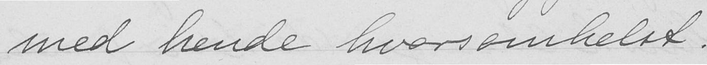med hende hvorsomhelst.
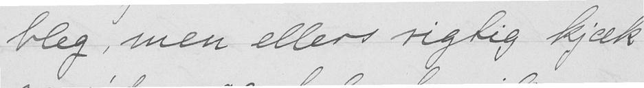bleg, men ellers rigtig kjæk
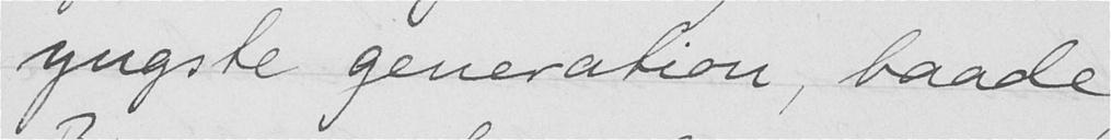yngste generation, baade
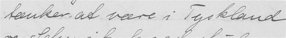tænker at være i Tyskland
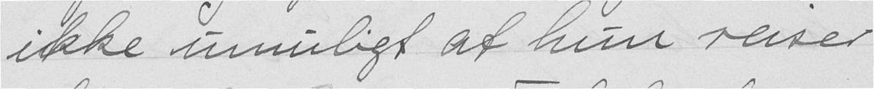ikke umuligt at hun reiser
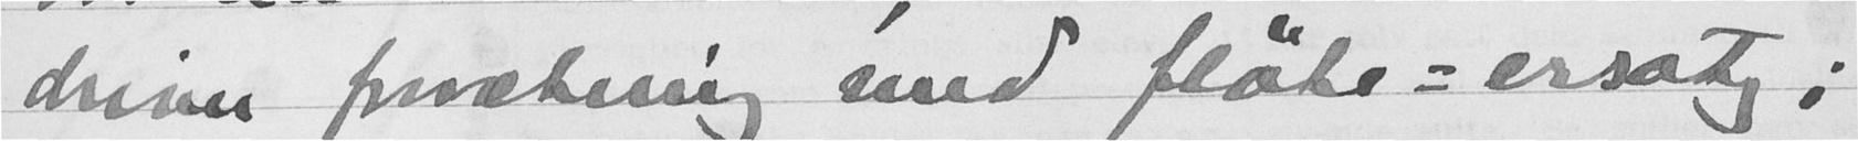driver forrætning med fløte-ersætg;
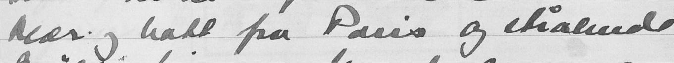klær og hatt fra Paris og strålende
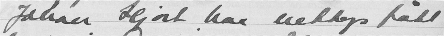Johan Hjort har nettop fått
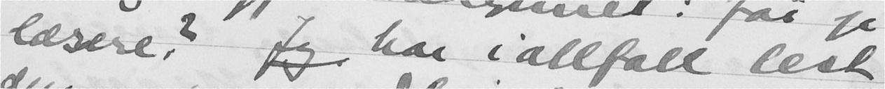læsere? Jeg har iallfall lest
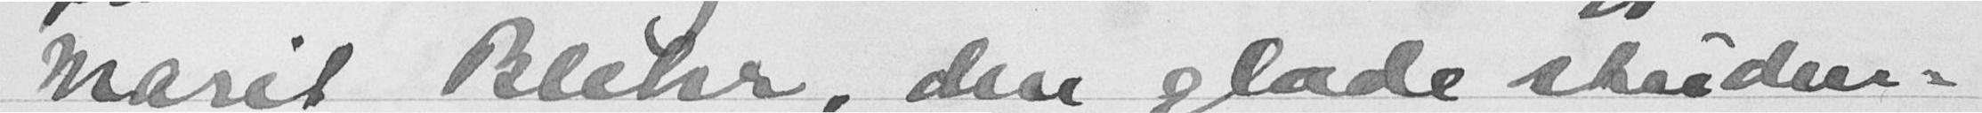Marit Blehr, den glade studen-
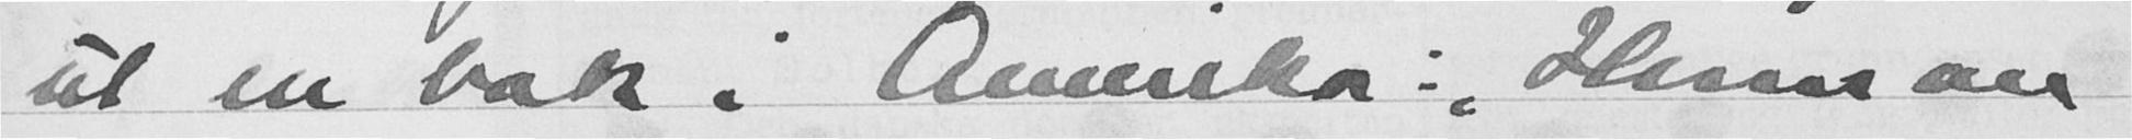ut en bok i Amerika:"Human
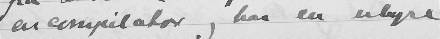en compilator og har en uhyre
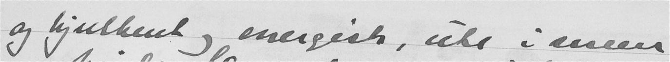og hjulbent og energisk, ute i sneen
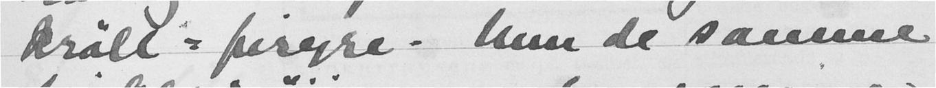krøll-frisyre. Men de samme
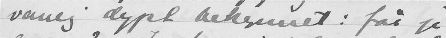vanlig dypt bekymret: får jeg
